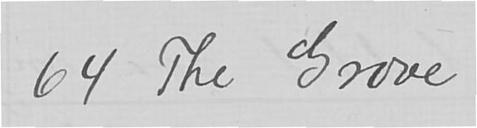64 The Grove
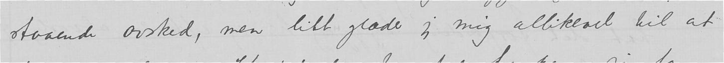staaende avsked, medn litt glæder jeg mig allikevel til at
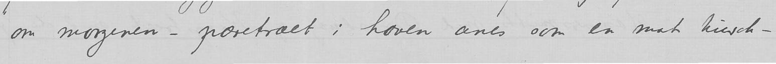om morgenen – pæretræet i haven anes som en mat tusch-
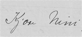Kjære Nini,
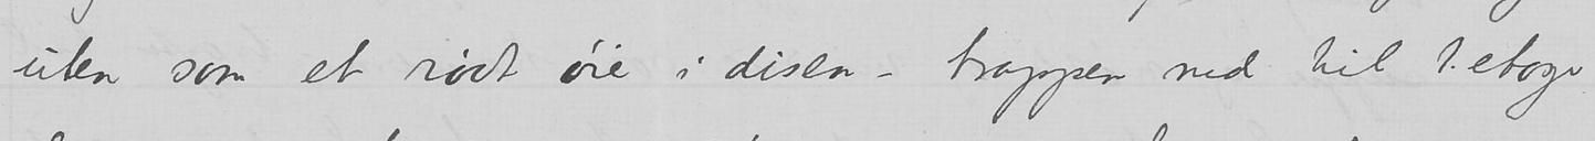uten som et rødt øie i disen – trappen ned til 1. etag
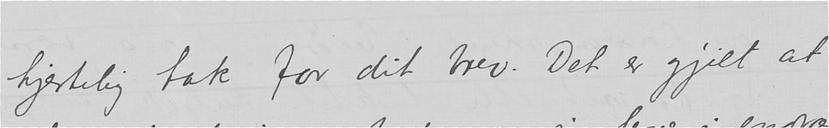hjertelig tak for dit brev. Det er gjilt at du er saa snil til at
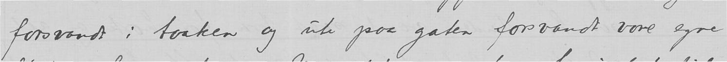forsvandt i taaken og ute paa gaten forsvandt vore egne
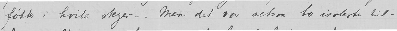føtter i hvite skyer –. Men det var altsaa to isolerte til-
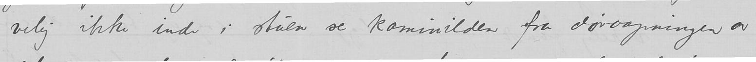stavelig ikke inde i stuen se kaminilden fra døraapningen av
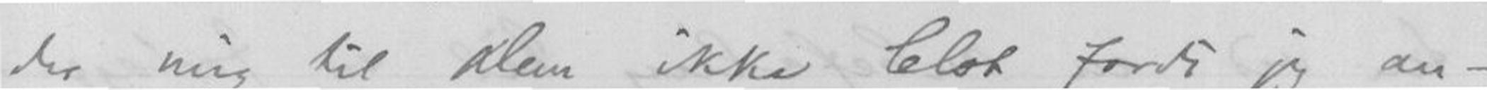der mig til Dem ikke blot fordi jeg an-
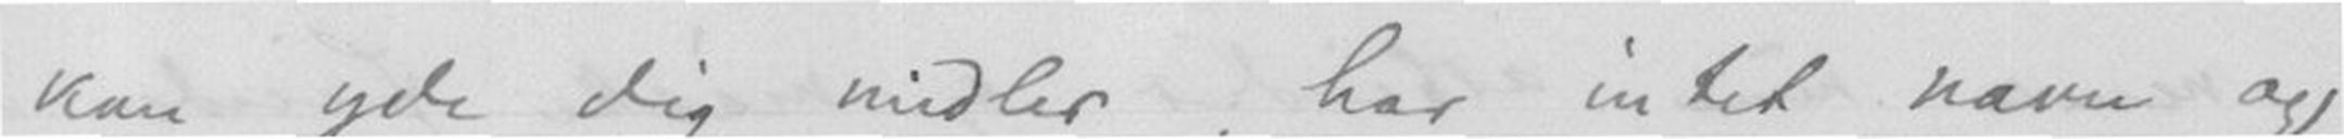kan yde dig midler, har intet navn og
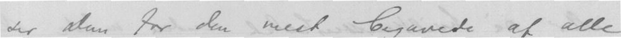ser Dem for den mest begavede af alle
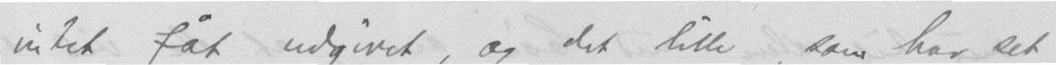intet fåt udgivet, og det lille, som har set
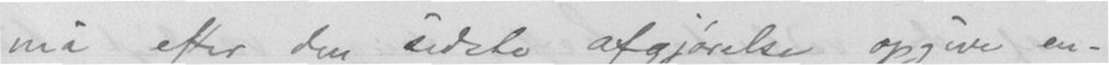må efter den sidste afgjørelse opgive en-
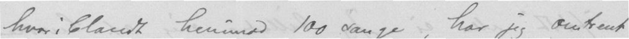hvoriblandt henimod 100 sange, har jeg omtrent
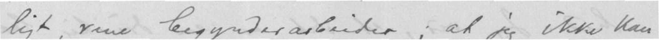ligt, rene begynderarbeider; at jeg ikke kan
